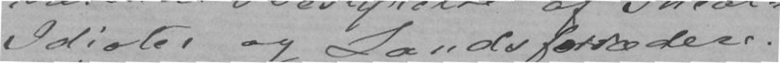Idioter og Landsforrædere.
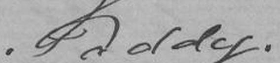til
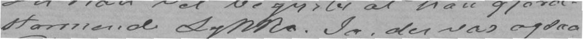stormende Lykke. Ja, der var ogsaa
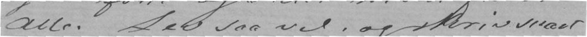Alle. Lev saa vel, og skriv snart
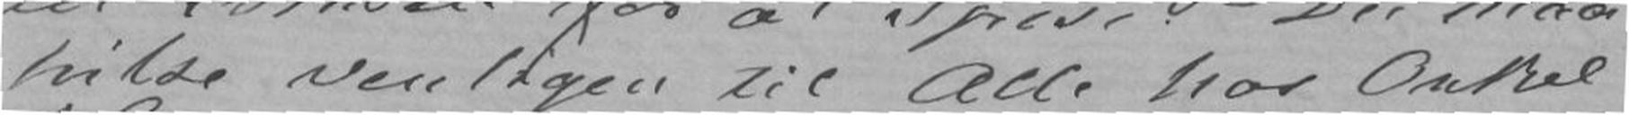hilse venligen til Alle hos Onkel
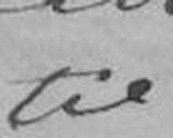Paddy.
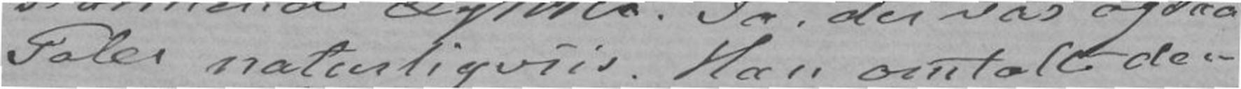Taler naturligviis. Han omtalte den
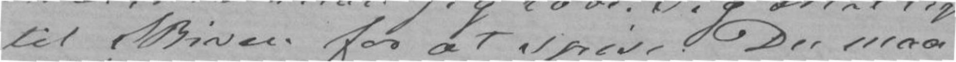til Skiven for at spise. Du maa
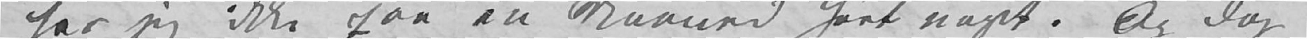har jeg ikke paa en Maaned hørt noget. Og dog
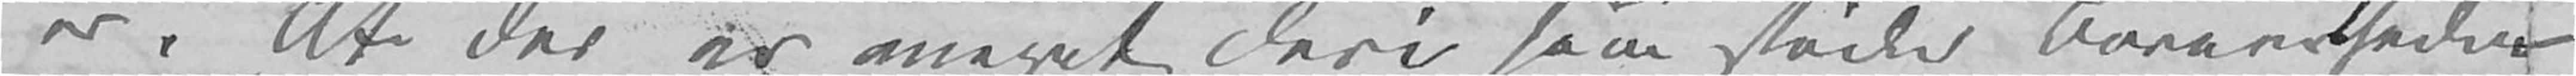er. At der er meget deri som støder Bornertheder
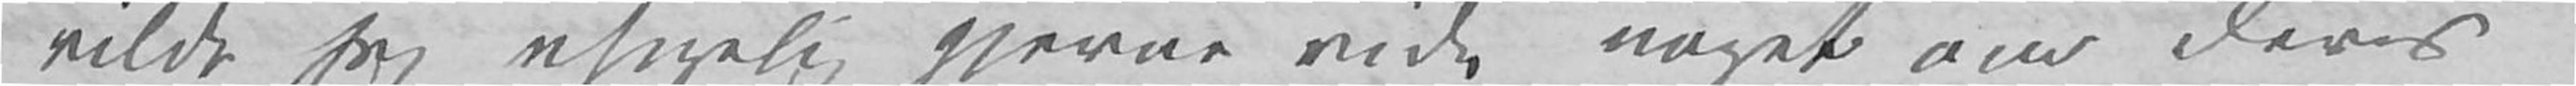vilde jeg usigelig gjerne vide noget om deres
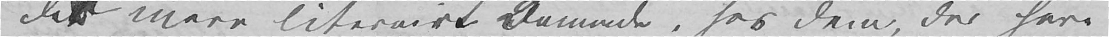det mere literairt Dannede, hos dem, der have
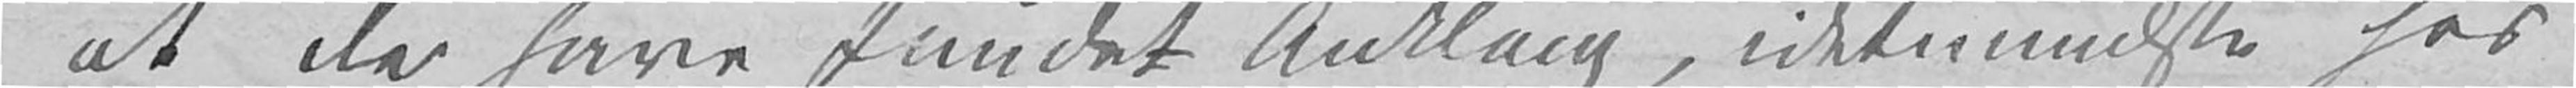at de have fundet Anklang, idetmindste hos
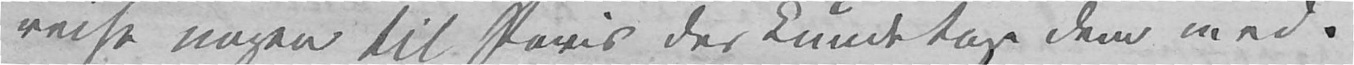reise nogen til Paris der kunde tage dem med?
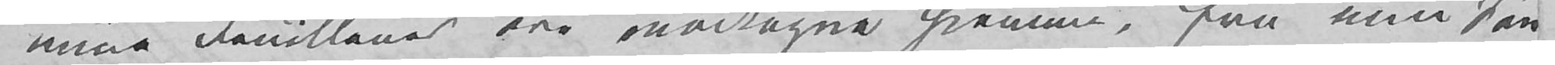mine Feuillener ere modtagne hjemme, fra min Søn
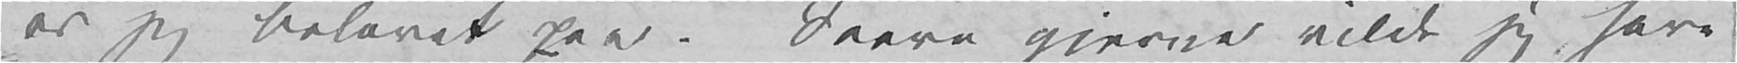er jeg belavet paa. Saare gjerne vilde jeg have
